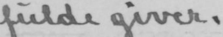fulde giver.
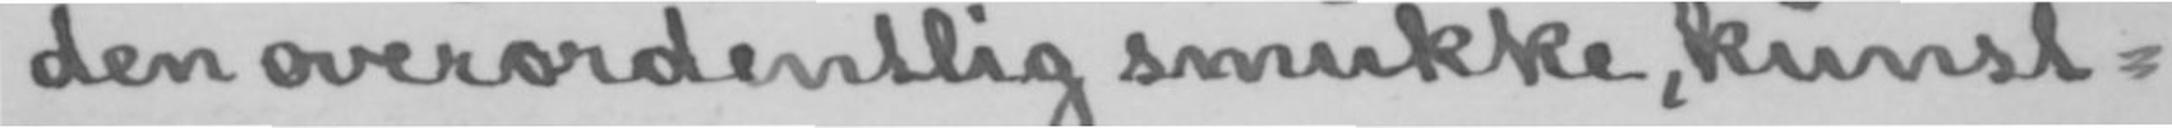den overordentlig smukke, kunst-
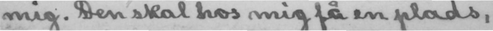mig. Den skal hos mig få en plads,
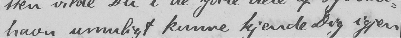havn umuligt kunne kjende Dig igjen
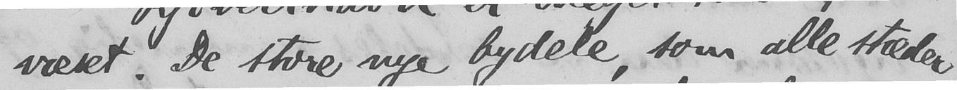væset. De store nye bydele, som alle stæder
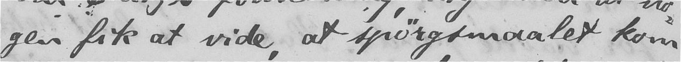gen fik at vide, at spørgsmaalet kom
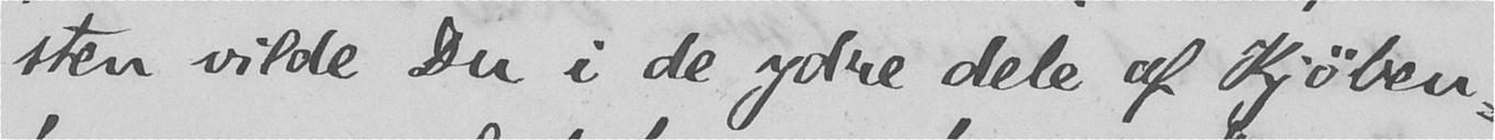sten vilde Du i de ydre dele af Kjøben-
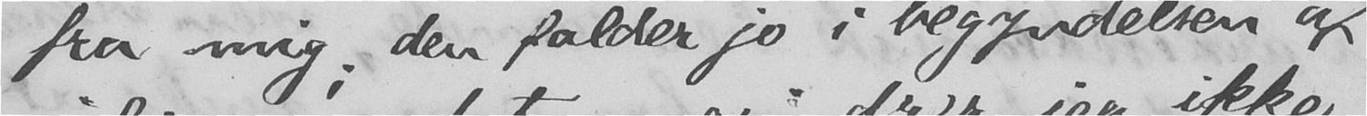fra mig; den falder jo i begyndelsen af
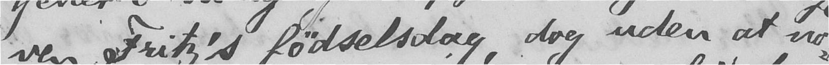ven Fritz's fødselsdag, dog uden at no-
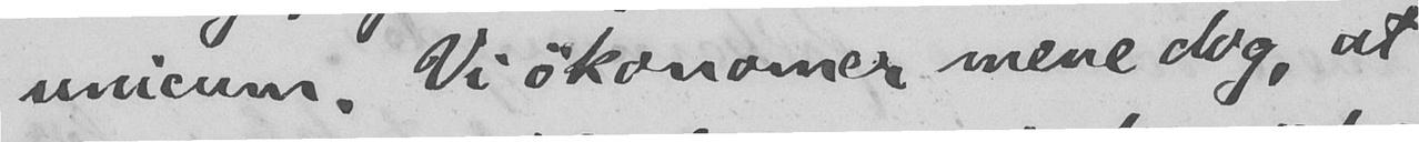unicum. Vi økonomer mene dog, at
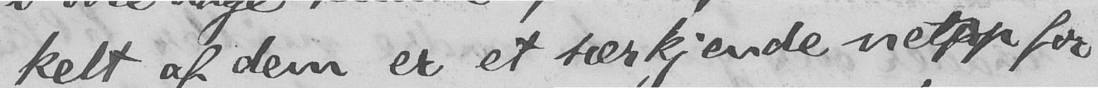kelt af dem er et særkjende netop for
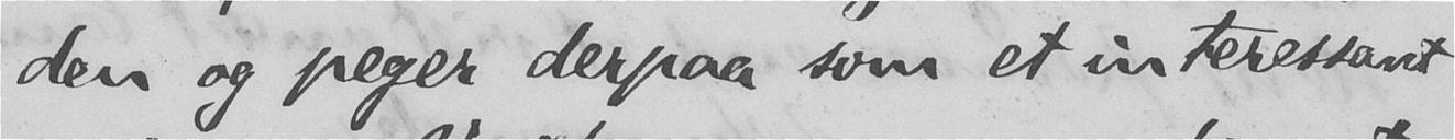den og peger derpaa som et interessant
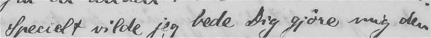Specielt vilde jeg bede Dig gjøre mig den
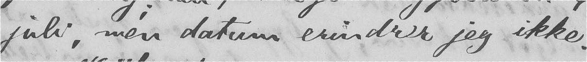juli, men datum erindrer jeg ikke.
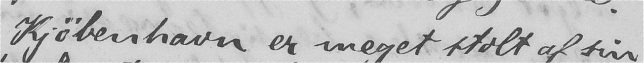Kjøbenhavn er meget stolt af sin
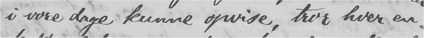i vore dage kunne opvise, tror hver en-
